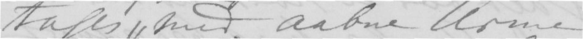tages, med aabne Arme
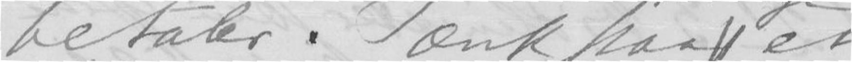betaler. Tænk paa et
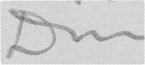Din
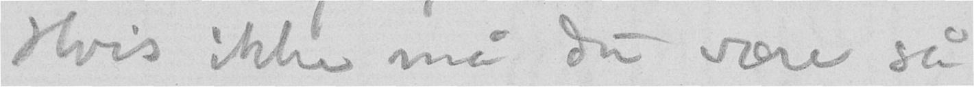Hvis ikke må du være så
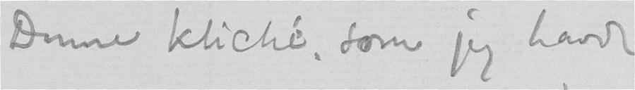Denne kliché, som jeg havde
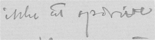ikke at opdrive.
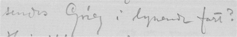sendes Grieg i lynende fart?
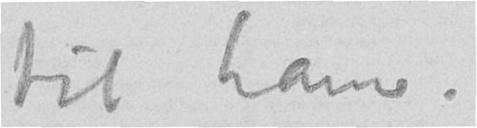til ham.
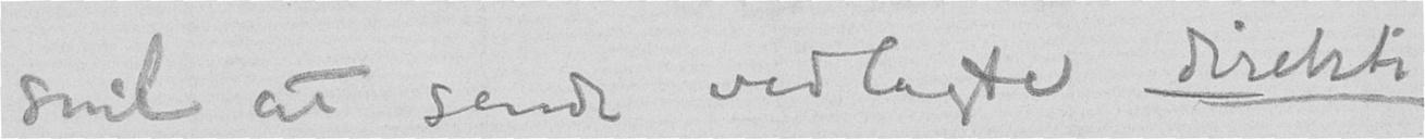snil at sende vedlagte direkte
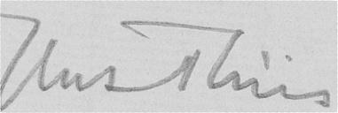Jens Thiis
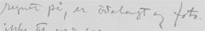synet på, er ødelagt og foto.
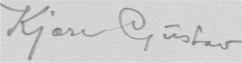Kjære Gustav
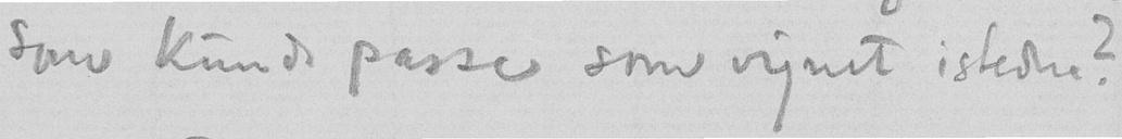som kunde passe som vignet isteden?
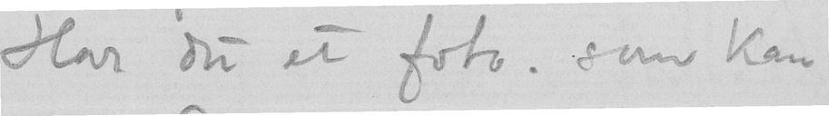Har du et foto, som kan
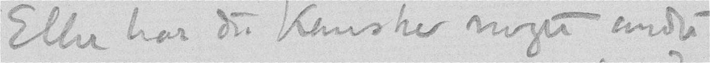Eller har du kanske noget andet
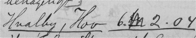Hvalby, Hov 6. 2.04
